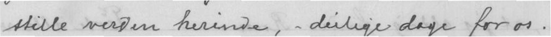stille verden herinde, - deilige dage for os.
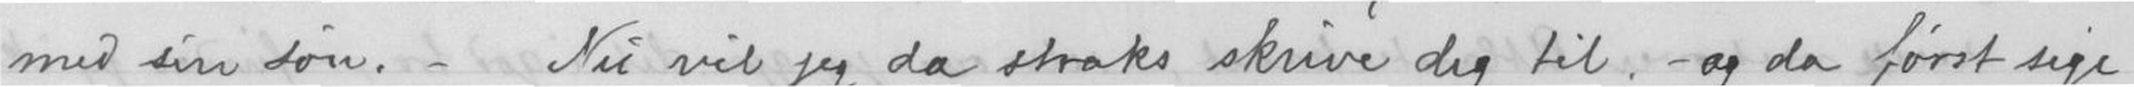med sin søn. - Nu vil jeg da straks skrive til. - og da først sige
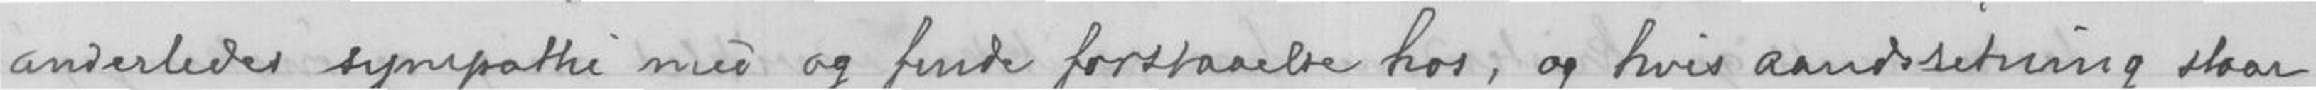enderledes sympathi med og finde forstaaelse hos, og hvis aandssetning staar
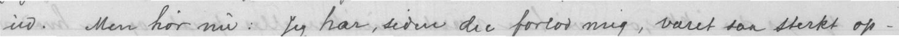ud. Men hør nu: Jeg har, siden du forlod mig, været saa sterkt op-
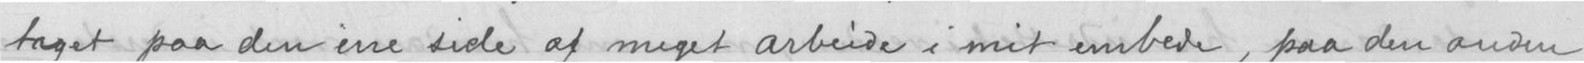taget paa den ene side af meget arbeide i mit embede, paa den anden
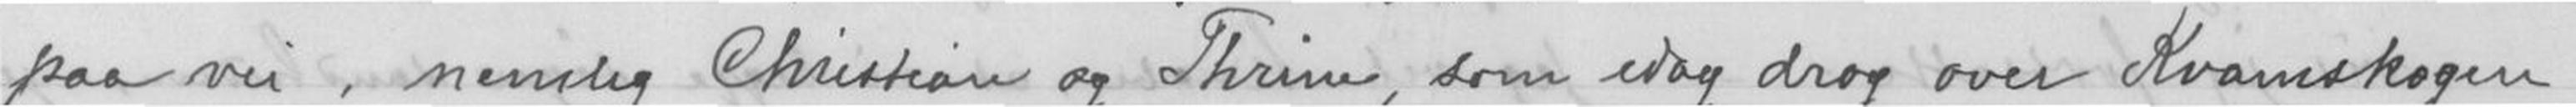paa vei, nemlig Christian og Thrine, som idag drog over Kvamsskogen
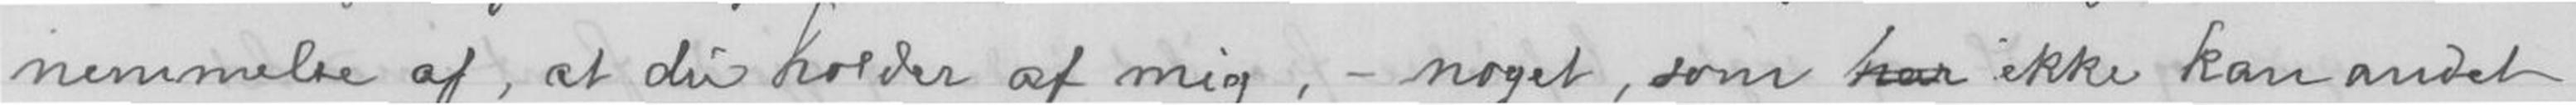nemmelse af, at du holder af mig, - noget, som ikke kan andet
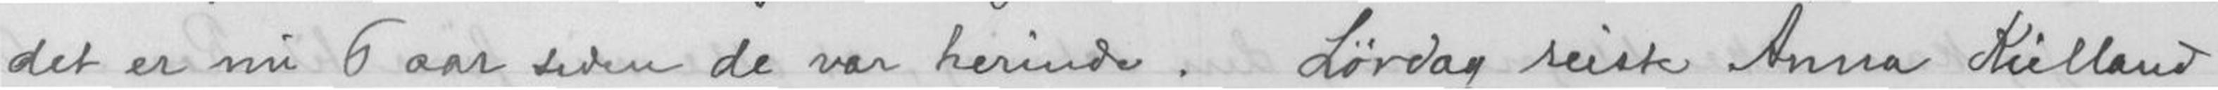det er nu 6 aar siden de var herinde. Lørdag reiste Anna Kielland
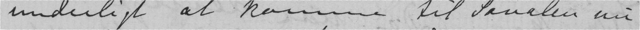underligt at komme til Savalen nu
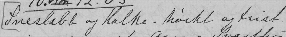Sneslabb og Holke. Mørkt og trist.
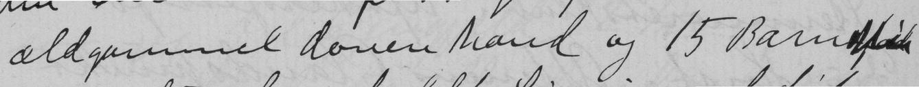ældgammel doven Mand og 15 Barn
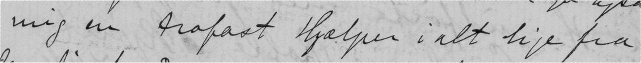mig en trofast Hjælper i alt lige fra
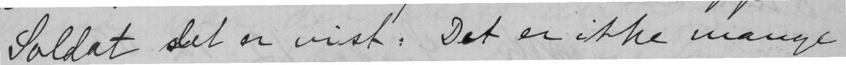Soldat det er vist: Det er ikke mange
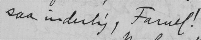saa inderlig, Farvel!
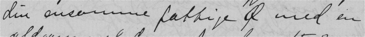din ensomme fattige Ø med en
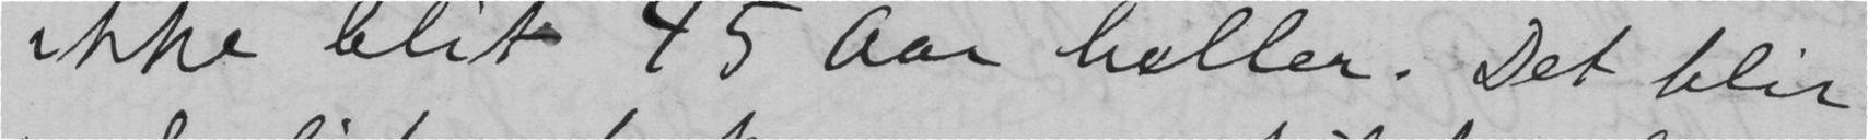ikke blit 45 aar heller. Det blir
